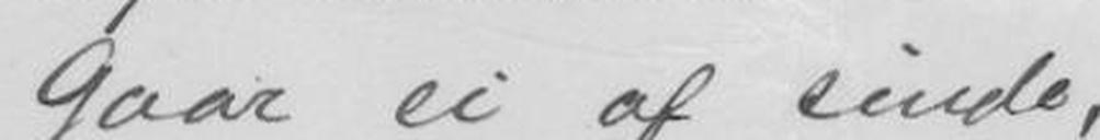Gaar ei af sinde,
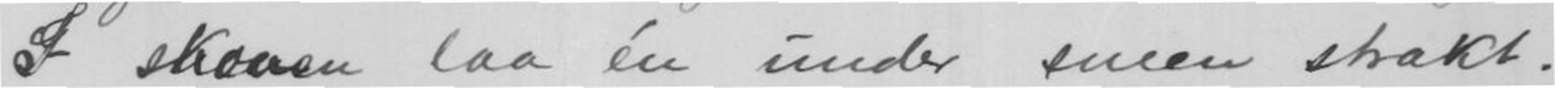I skoven laa en under sneen strakt.
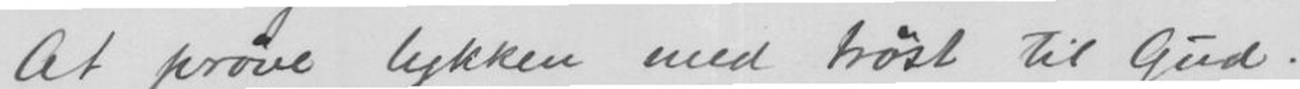At prøve lykken med trøst til Gud.
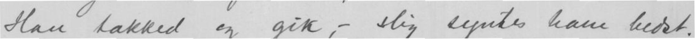Han takket og gik,- slig syntes ham bedst.
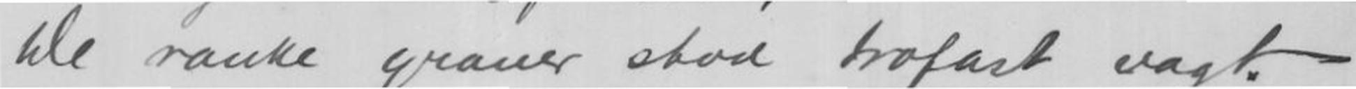De ranke graner stod trofast vagt.
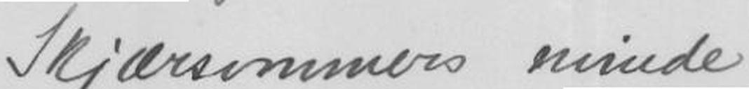Skjærsommers minde
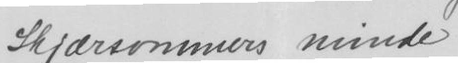Skjærsommers minde
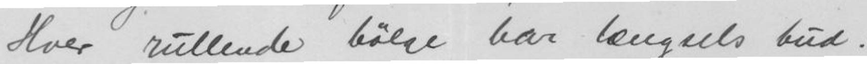Hver rullende bølge bar længsels bud.
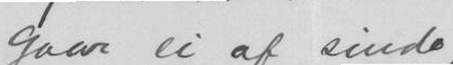Gaar ei af sinde,
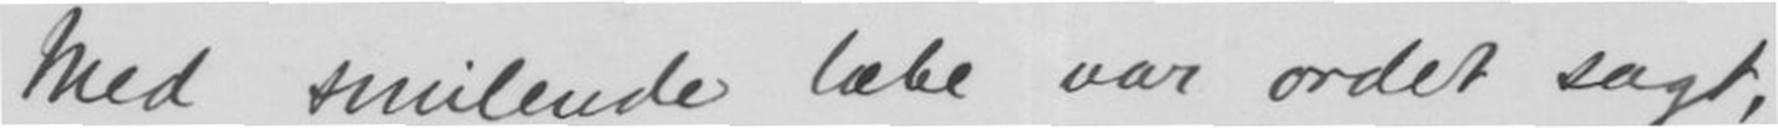Med smilende læbe var ordet sagt,
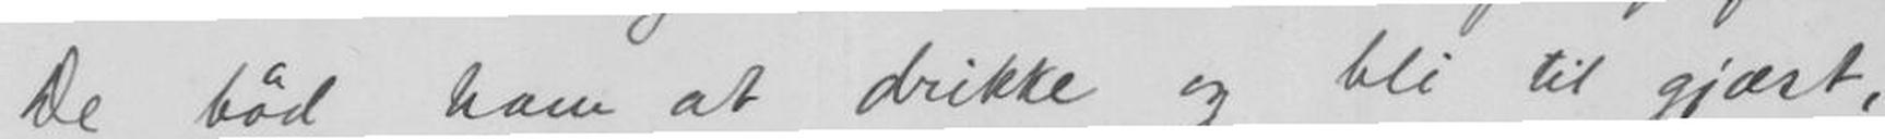De bød ham at drikke og bli til gjæst, -
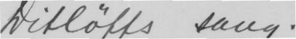Ditløffs sang.
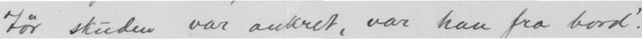Før skuden var ankret, var han fra bord!
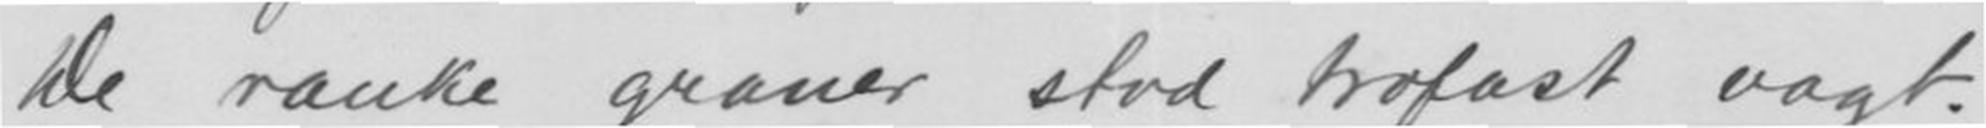De ranke graner stor trofast vagt.
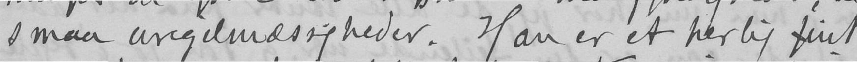smaa uregelmæssigheder. Han er et herlig fint
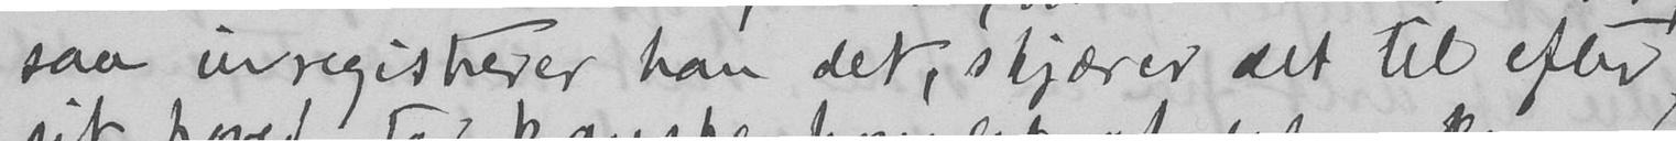saa inregisterer han det, skjærer det til efter
